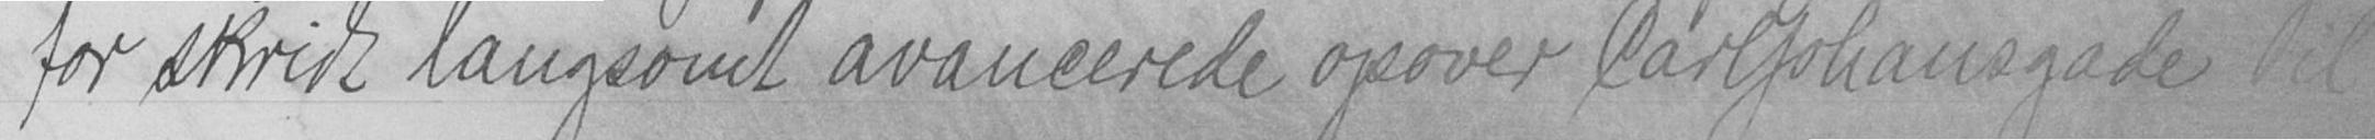for skridt langsomt avancerede opover Carljohansgade til
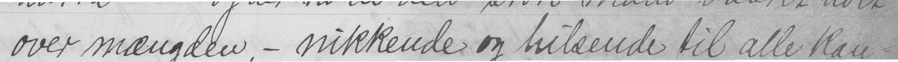over mængden, - nikkende og hilsende til alle kan-
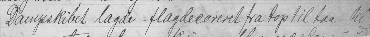Dampskibet lagde - flagdecoreret fra top til taa - til
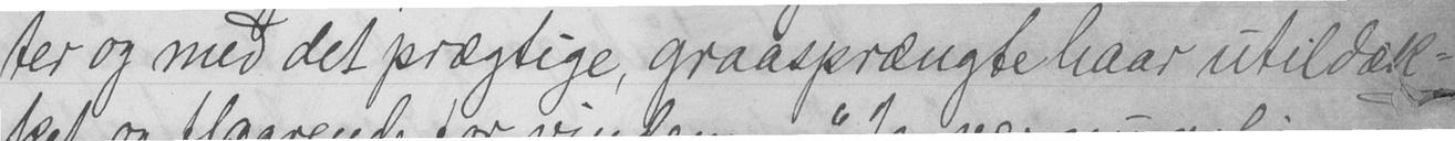ter og med det prægtige, graasprængte haar utildæk-
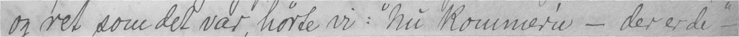og ret som det var, hørte vi: "nu kommer'n - der er de" -
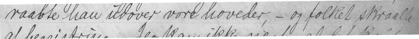raabte han udover vore hoveder, - og folket skraalte
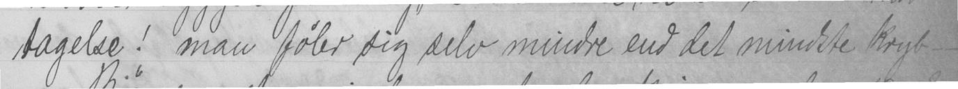tagelse! man føler sig selv mindre end det mindste kryb - -
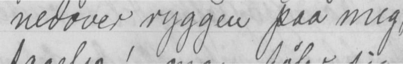nedover ryggen paa mig
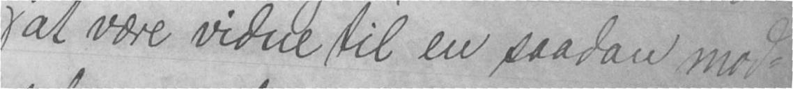at være vidne til en saadan mod-
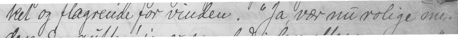ket og flagrende for vinden. "Ja, vær nu rolige med
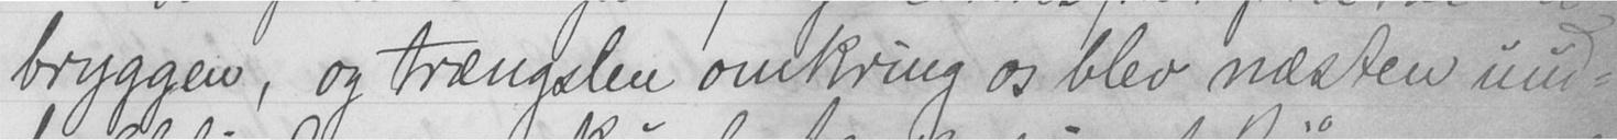bryggen, og trængslen omkring os blev næsten uud-
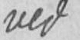ved
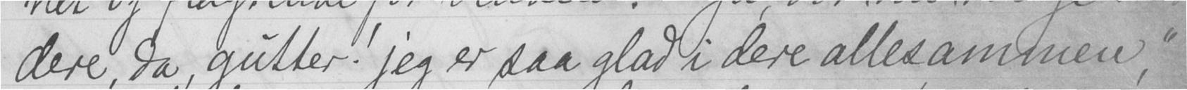dere, da, gutter! jeg er saa glad i dere allesammen,"
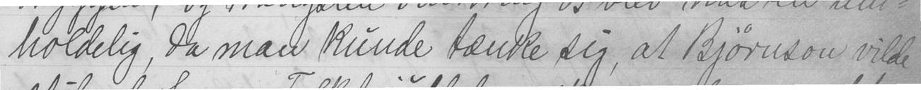holdelig, da man kunde tænke sig, at Bjørnson vilde
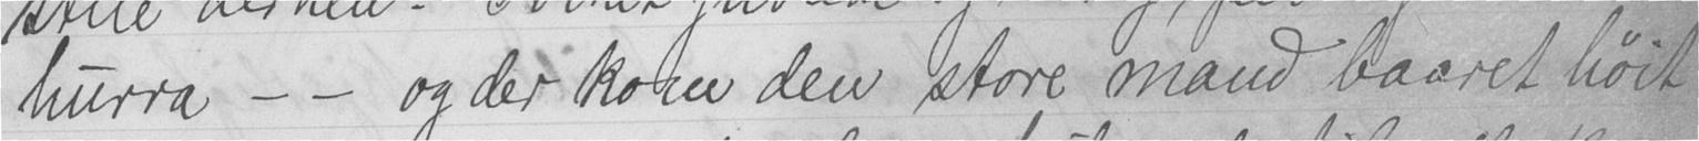hurra - - og der kom den store mand baaret høit
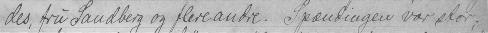des, fru Sandberg og flere andre. Spændingen var stor;
